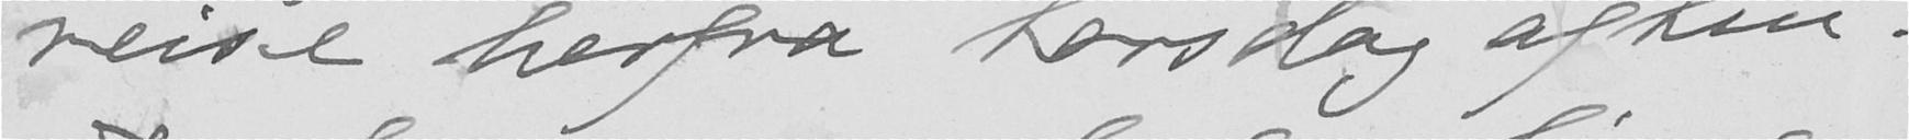reise herfra torsdag aften.
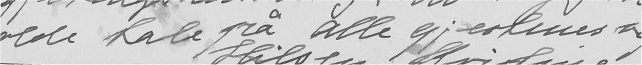holde tale på alle gjestenes vegne.
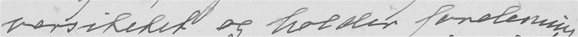versitetet og holder forelesning.
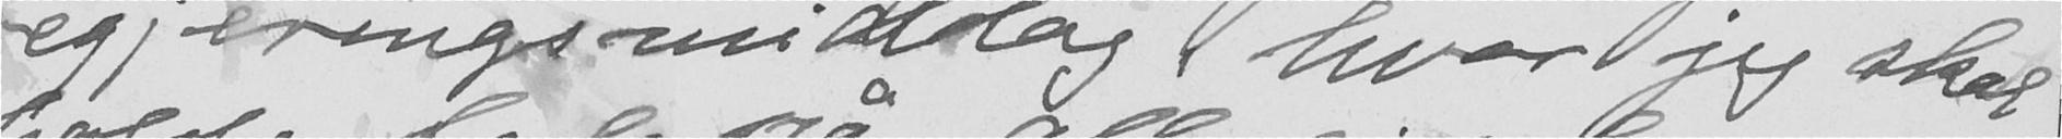regjeringsmiddag hvor jeg skal
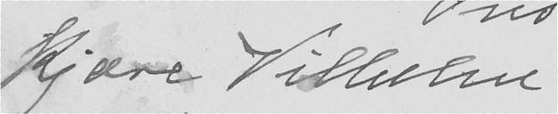Kjære Vilhelm
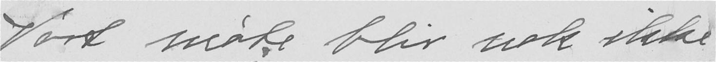Vårt møte blir nok ikke
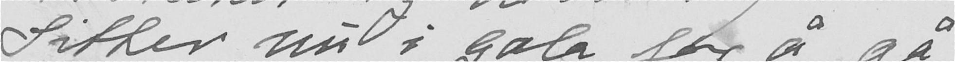Sitter nu i gala for å gå i
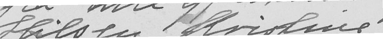Hilsen Kristine
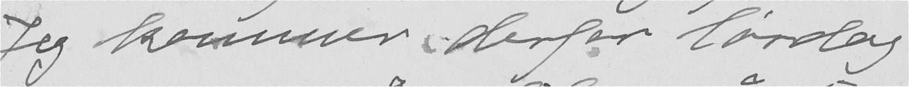Jeg kommer derfor lørdag
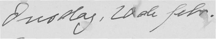Onsdag, 20de febr.
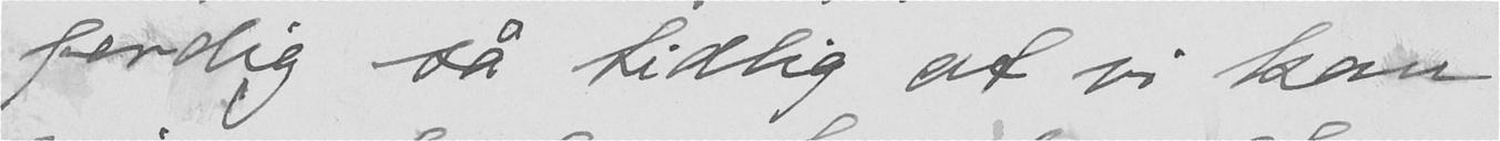ferdig så tidlig at vi kan
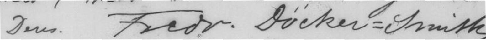Deres Freds. Döcker=Smith
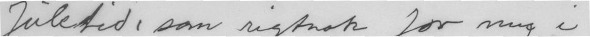Juletid, som rigtnok for mig i
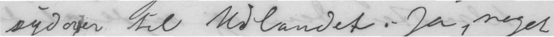syd over til Udlandet. Ja, noget
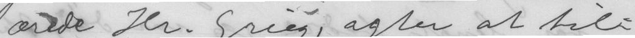ærede Hr. Grieg, agter at til-
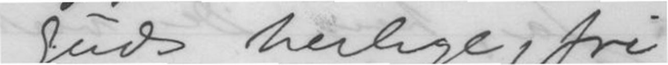Guds herlige, fri
